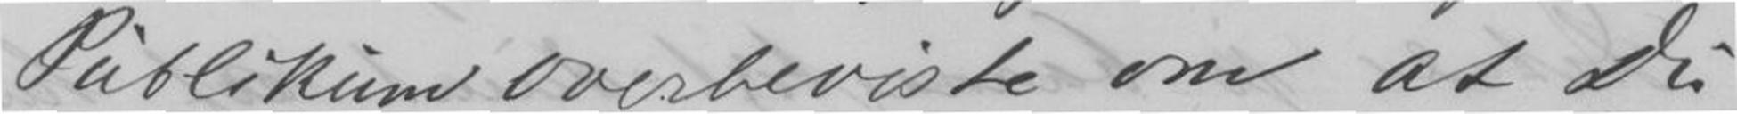Publikum overbeviste om at Du
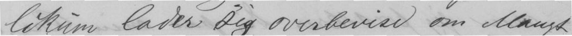ikum lader sig overbevise om Mangt
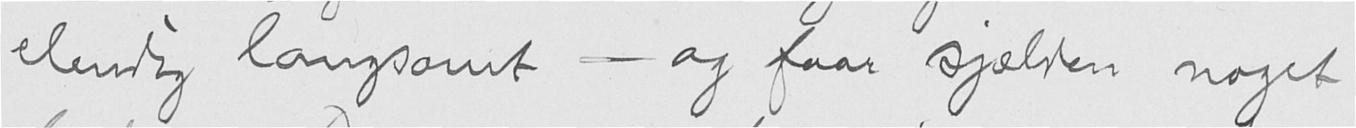elendig langsomt - og faar sjælden noget
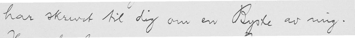har skrevet til dig om en Byste av mig.
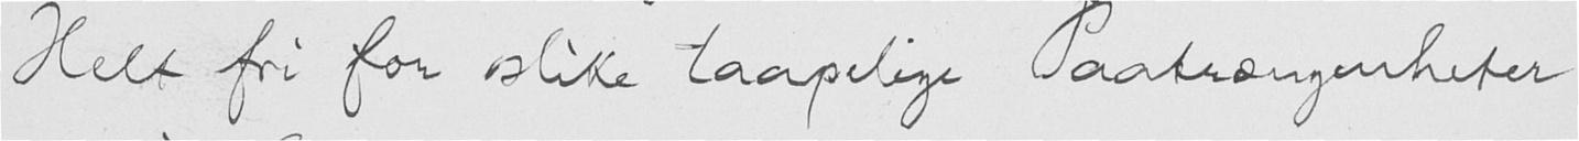Helt fri for slike taapelige Paatrængenheter
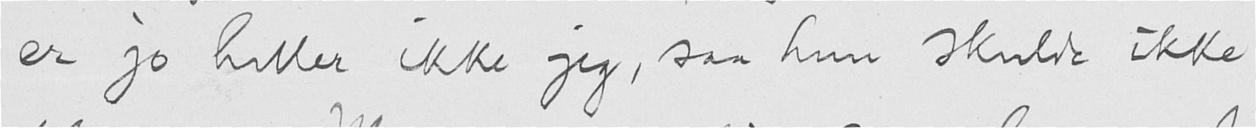er jo heller ikke jeg, saa hun skulde ikke
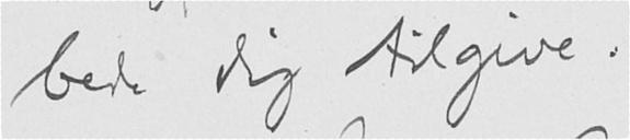bede dig tilgive.
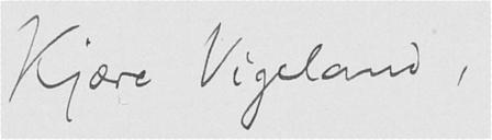Kjære Vigeland,
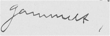gammelt,
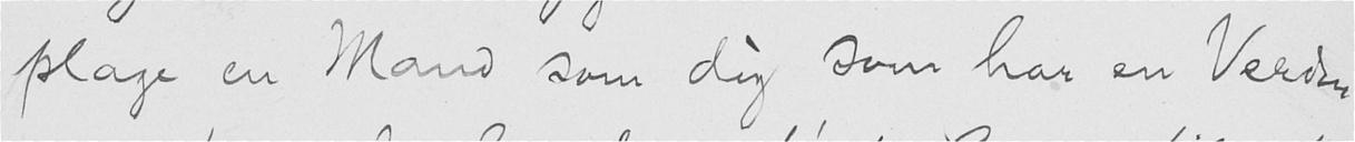plage en Mand som dig som har en Verden
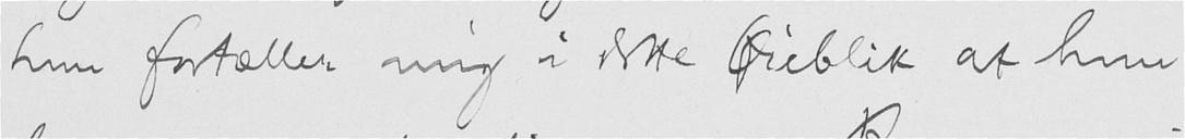hun fortæller mig i dette Øieblik at hun
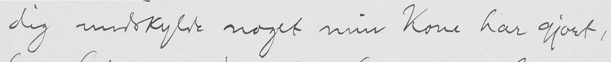dig undskylde noget min Kone har gjort,
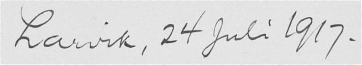Larvik, 24 Juli 1917.
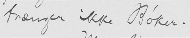trænger ikke Bøker.
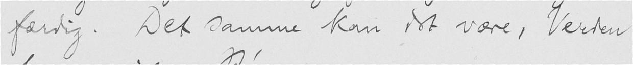færdig. Det samme kan det være, Verden
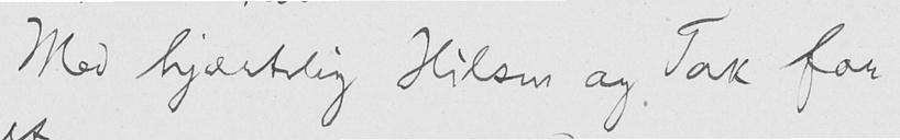Med hjærtelig Hilsen og Tak for
